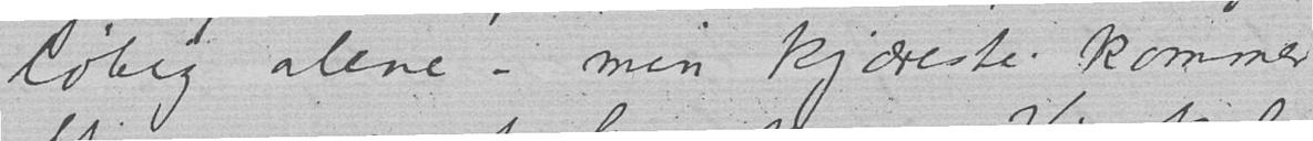løbig alene - min kjæreste kommer
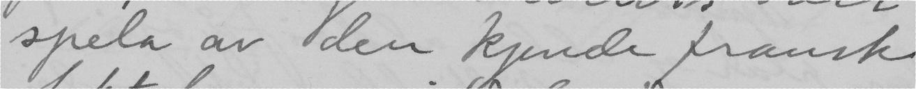spela av den kjende fransk
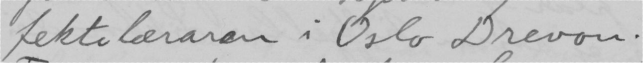fektelæraren i Oslo Drevon.
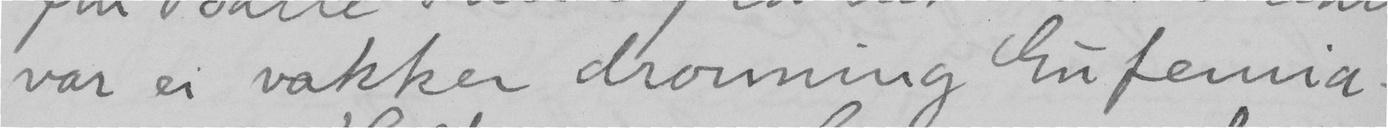var ei vakker dronning Eufemia –
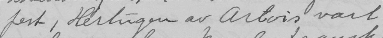fest, Hertugen av Artois vart
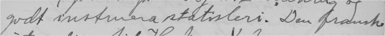godt instruera statisteri. Den franske
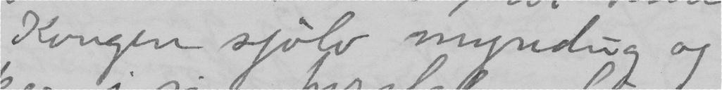Kongen sjölv myndug og
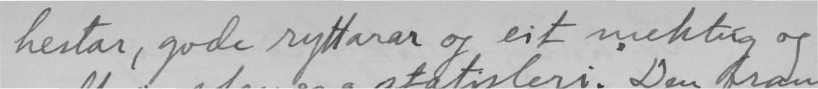hestar, gode ryttarar og eit mektig og
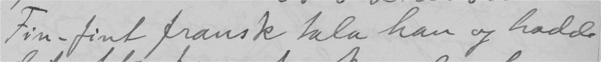Fin-fint fransk tala han og hadde
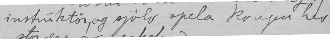instruktör, og sjölv spela kongen hev
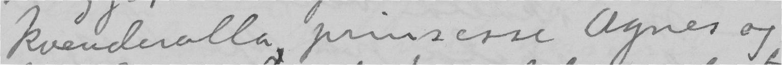kvenderolla, prinsesse Agnes og
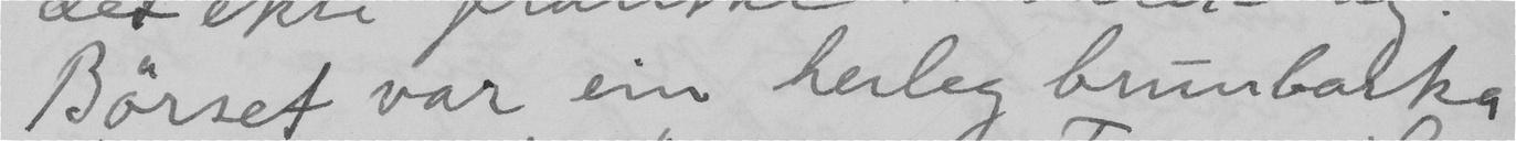Børset var ein herleg brunbarka
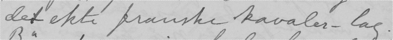det ekte franske kavaler-lag.
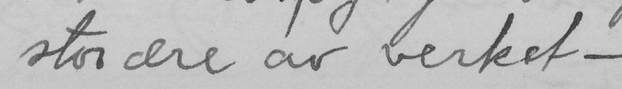stor ære av verket —
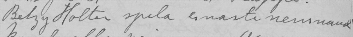Betzy Holter spela einaste nemnand
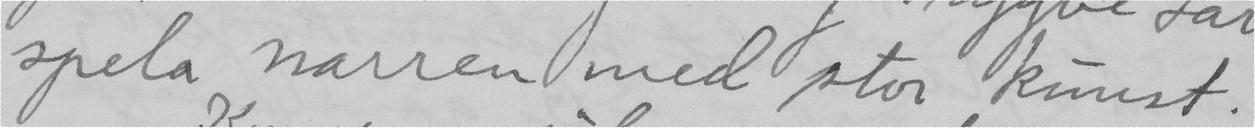spela narren med stor kunst.
